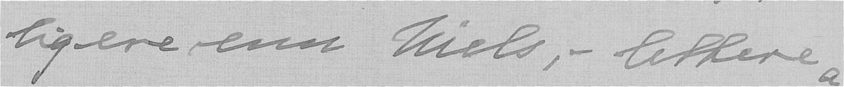ligere enn Niels, - lettere og
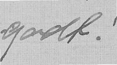godt!
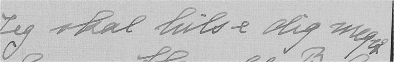Jeg skal hilse dig meget
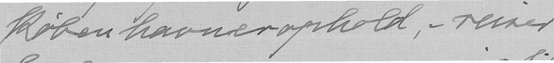Københavnerophold - reiser
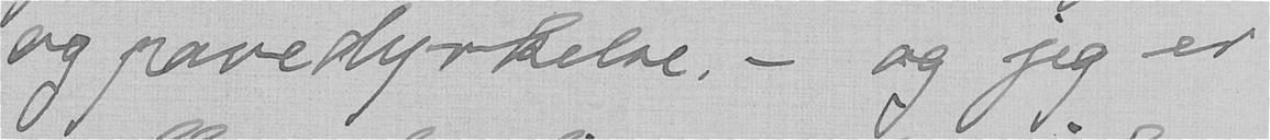og pavedyrkelse, - og jeg er
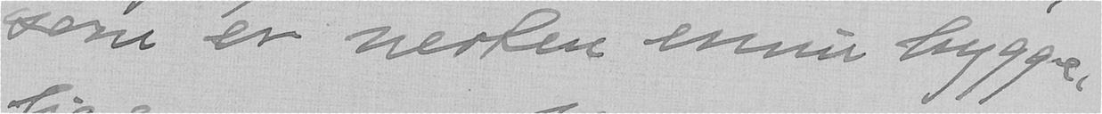som er nesten ennu hygge-
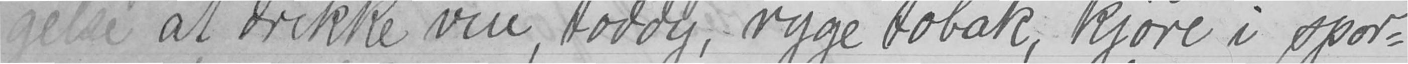gelse at drikke vin, toddy, ryge tobak, kjøre i spor-
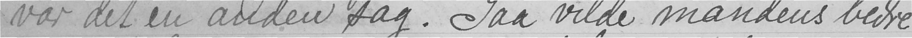var det en anden sag. Saa vilde mandens bedre
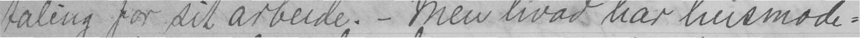taling for sit arbeide. - Men hvad har husmode-
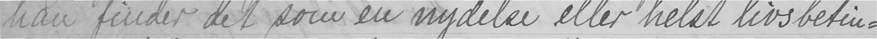han finder det som en nydelse eller helst livsbetin-
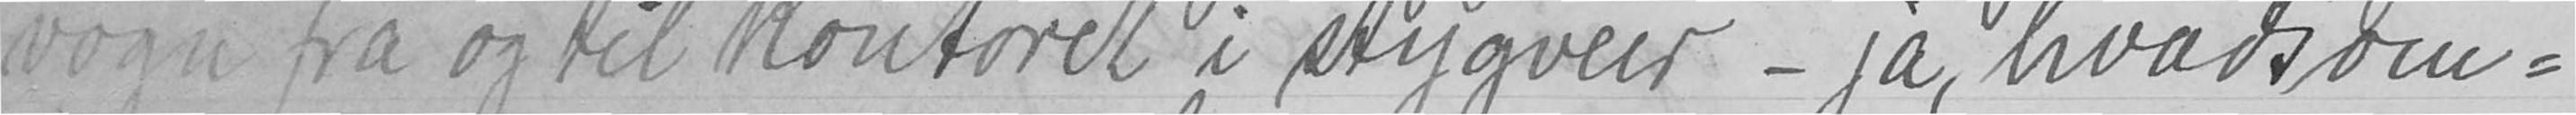vogn fra og til kontoret i stygveir - ja, hvadsom-
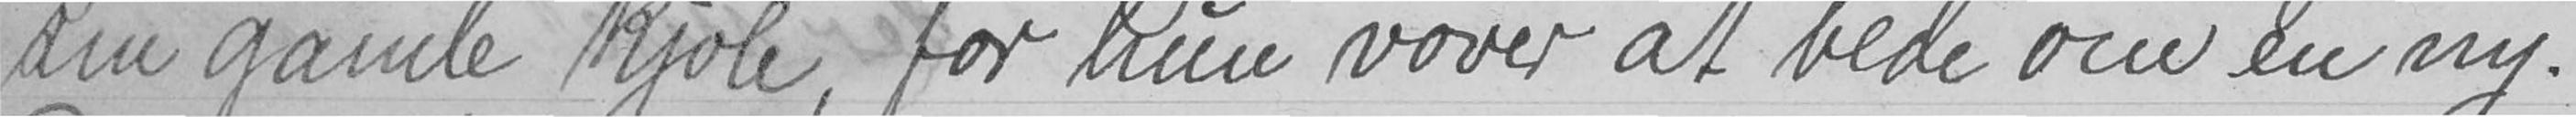sin gamle kjole, for hun vover at bede om en ny.
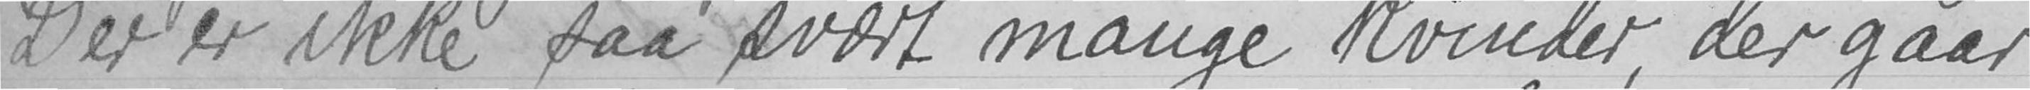Der er ikke saa svært mange kvinder, der gaar
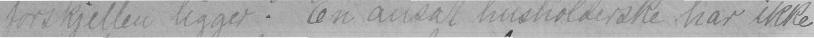forskjellen ligger? En ansat husholderske har ikke
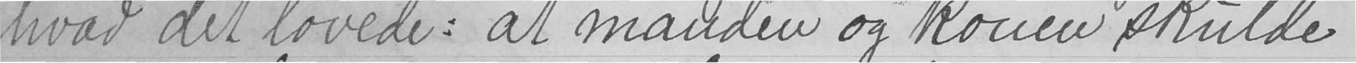hvad det lovede: at manden og konen skulde
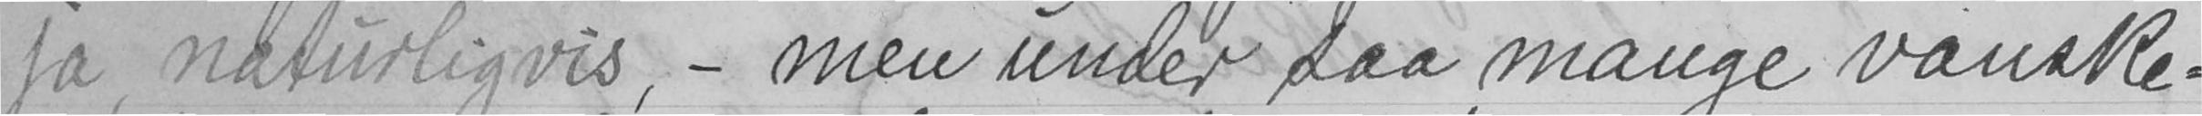ja, naturligvis, - men under saa mange vanske-
